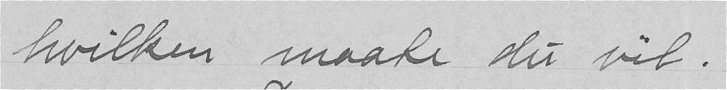hvilken maate du vil.
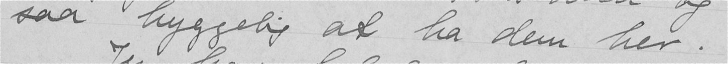saa hyggelig at ha dem her.
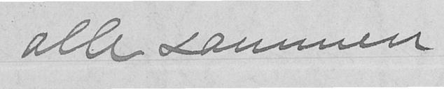alle sammen
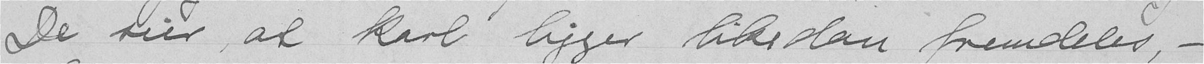De sier, at Karl ligger likedan fremdeles, -
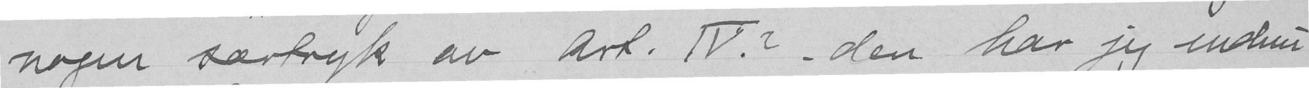nogen særtryk av art. IV? - den har jeg endnu
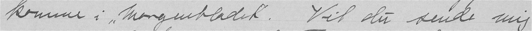komme i Morgenbladet. Vil du sende mig
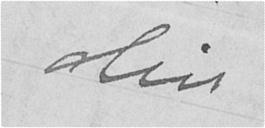din
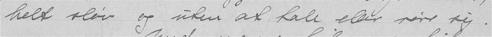helt sløv og uten at tale eller røre sig.
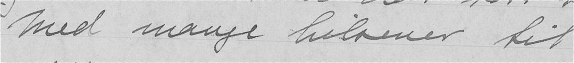Med mange hilsener til
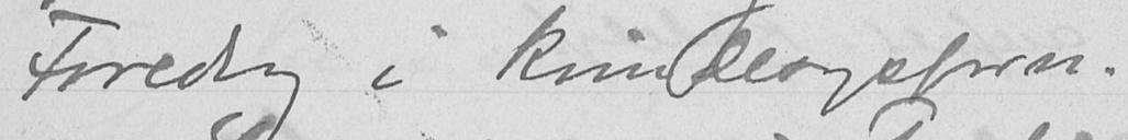Foredrag i kvindesagsforn.
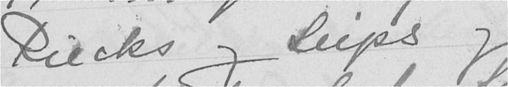Riecks og Seips og
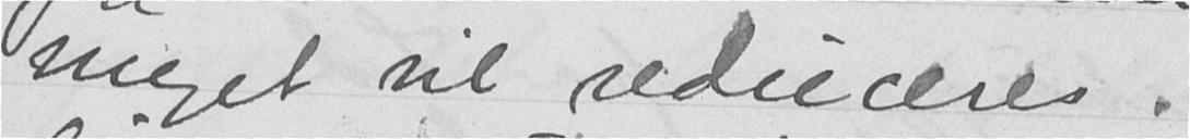meget vil reduceres.
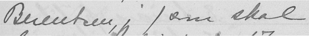Berentsen, jeg) som skal
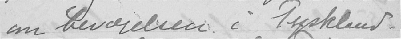om bevægelsen i Tyskland.
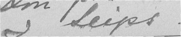og Seips.
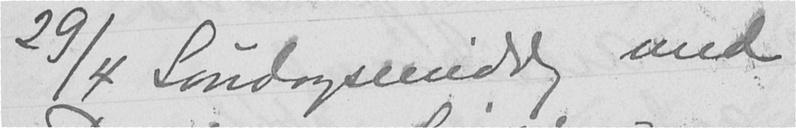29/4 Søndagsmiddag med
